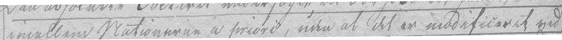mellem Nationerne a priori, uden at det er modificeret ved
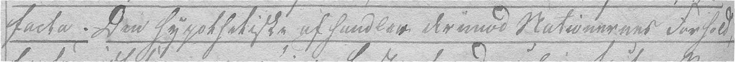facta. Den hypothetiske afhandler derimod Nationernes Forhold
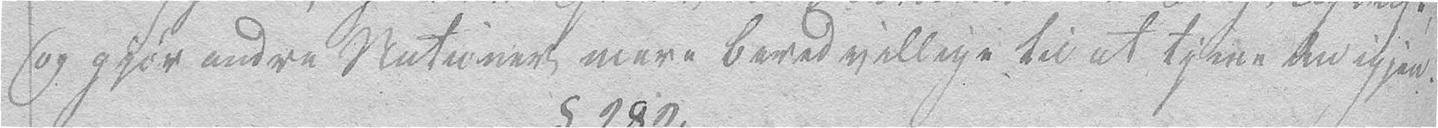og gjør andre Nationer mere beredvillige til at tjene den igjen.
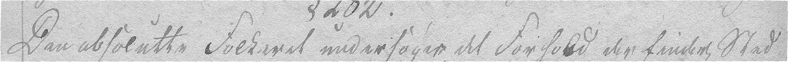Den absolutte Folkeret undersøger det Forhold der finder Sted
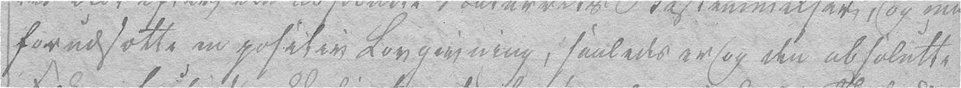forudsætte en positiv Lovgivning, saaledes er og den absolutte
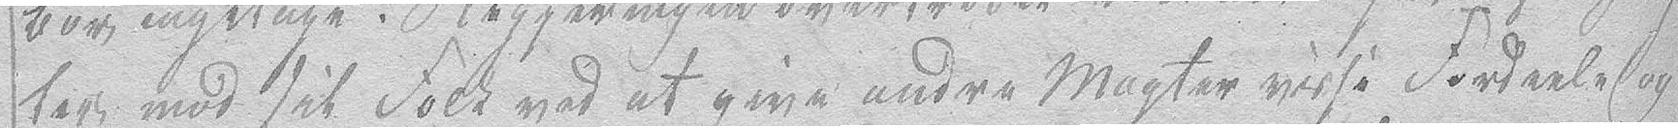ter mod sit Folk ved at give andre Magter visse Fordeele og
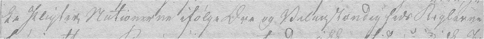ke Pligter Nationerne ifølge Ære og Velanstændigheds Reglerne
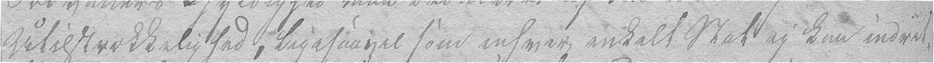Utilstrækkelighed, ligesaavel som enhver enkelt Stat ey kan indret-
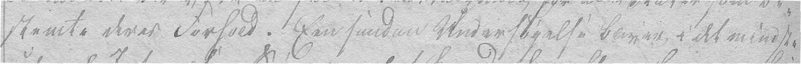stemte deres Forhold. En saadan Undersøgelse bliver i det mindste
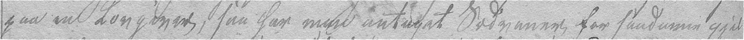paa en Lovgiver, saa har man antaget Sædvaner for saadanne gjæl-
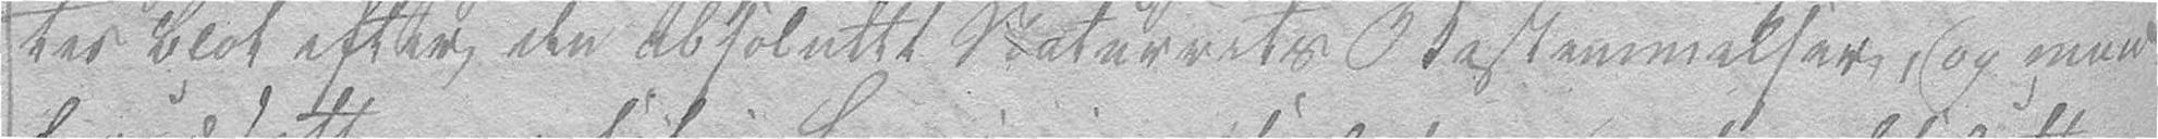tes blot efter den absolutte Naturrets Bestemmelser, og maa
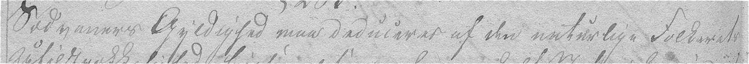Sædvaners Gyldighed maa deduceres af den naturlige Folkerets
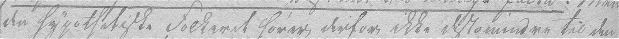den hypothetiske Folkeret hører derfor ikke destomindre til den
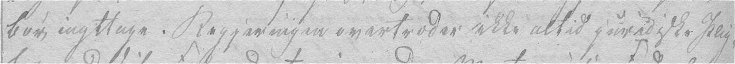bør iagttage. Regjeringen overtræder ikke altid juridiske Plig-
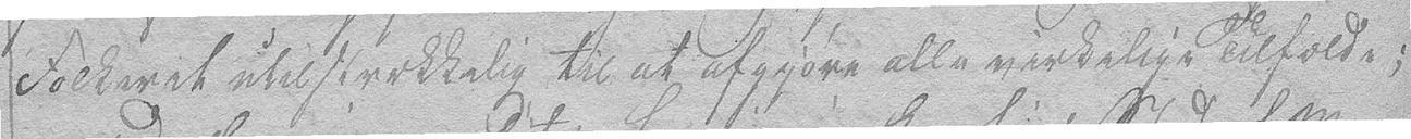Folkeret utilstrækkelig til at afgjøre alle virkelige Tilfælde;
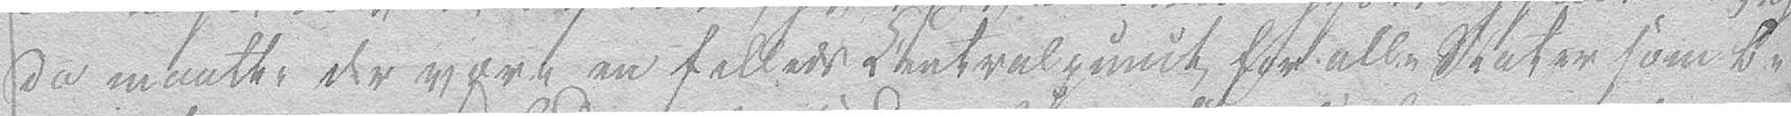da maatte der være en fælleds Controlpunct for alle Stater som be-
